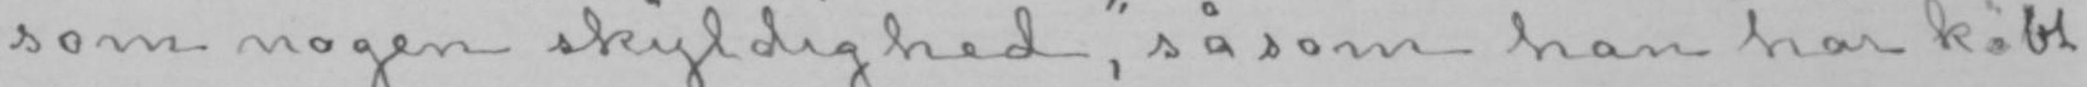som nogen skyldighed, «såsom han har købt
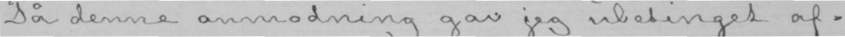På denne anmodning gav jeg ubetinget af-
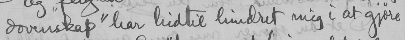dovenskap" har hidtil hindret mig i at gjøre
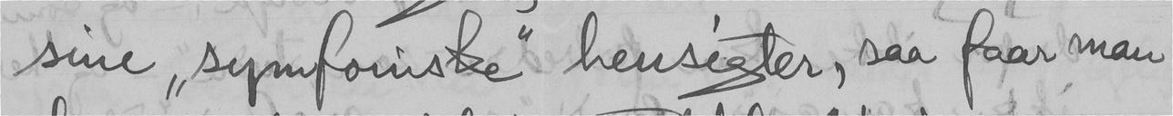sine "symfoniske" hensigter, saa faar man
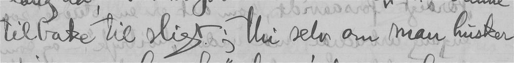tilbake til sligt; thi selv om man husker
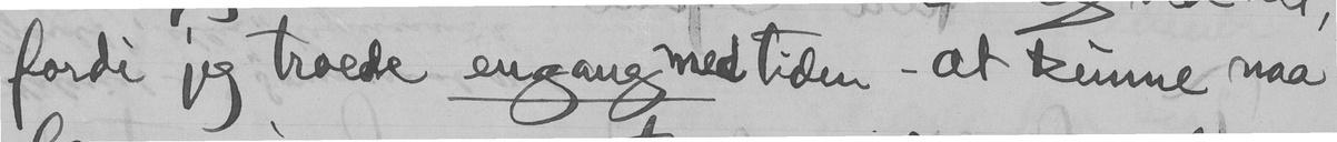fordi jeg troede engang med tiden - at kunne naa
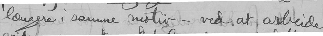længere i samme motiv - ved at arbeide
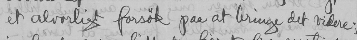et alvorligt forsøk paa at bringe det videre;
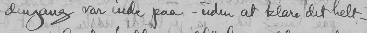dengang var inde paa - uden at klare det helt, -
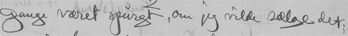gange været spurgt, om jeg vilde sælge det;
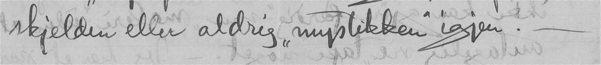skjelden eller aldrig "mystikken" igjen. -
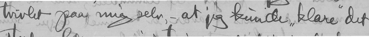tvivlet paa mig selv, - at jeg kunde "klare" det
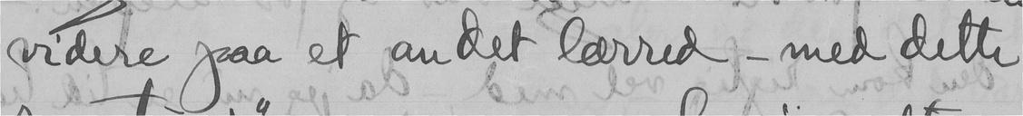videre paa et andet lærred - med dette
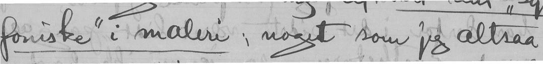foniske" i maleri; noget som jeg altsaa
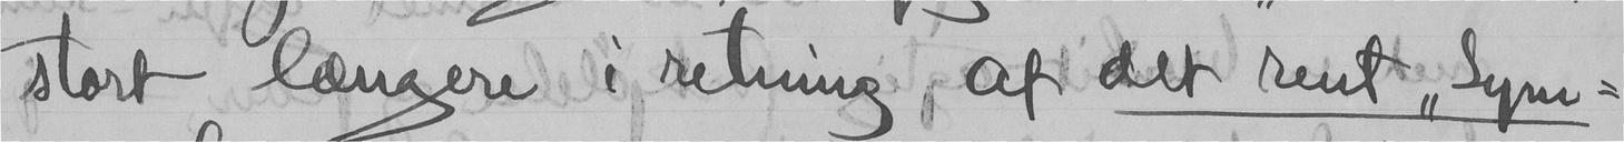stort længere i retning af det rent "Sym-
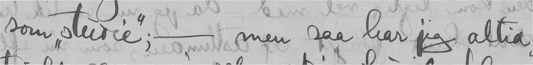som "studie"; - men saa har jeg altid
